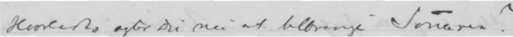Hvorledes agter du nu at tilbringe Somaren?
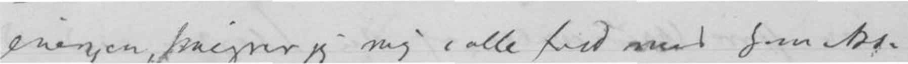eningen smigrer jeg mig i alle fald med som Art-
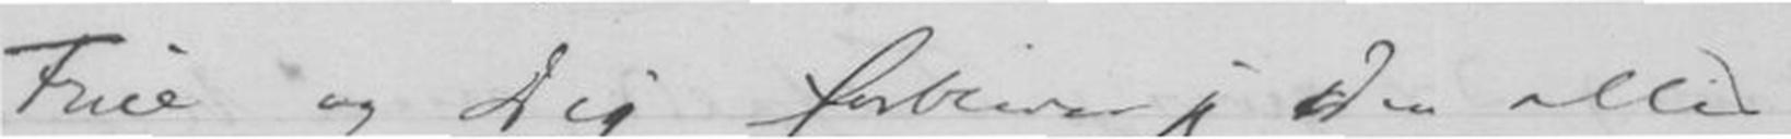Frue og Dig forbliver jeg Din altid
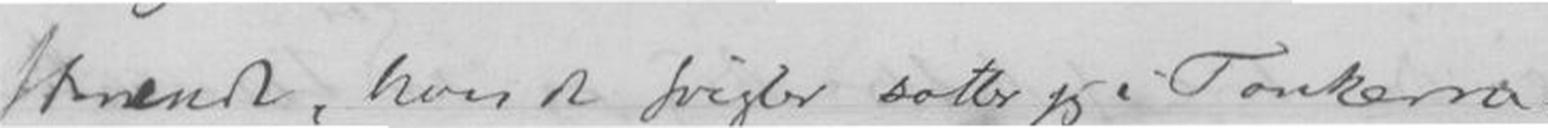strænde, hvor de svigter sætter jeg i Tankerne
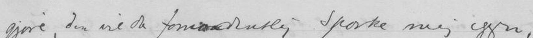gjøre, den vil da formodentlig Sparke mig igjen,
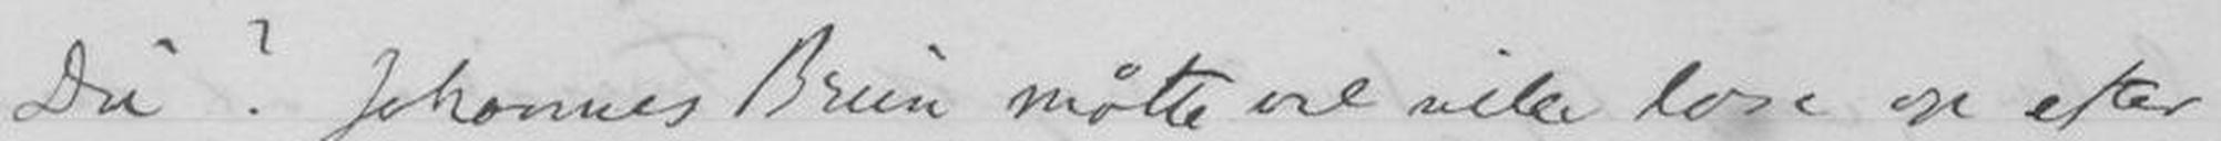Du? Johannes Brun Måtte vel ville læse op efter
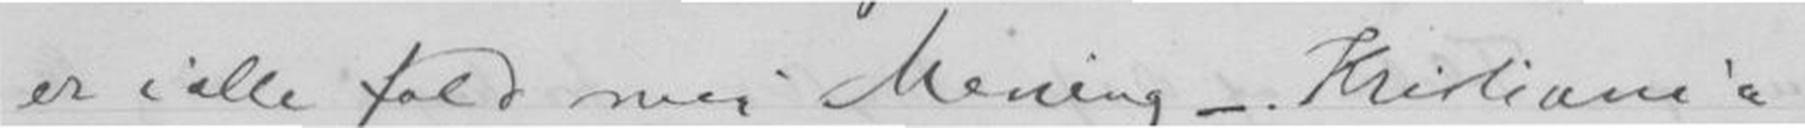er i alle fald min Mening - Kristiania skal
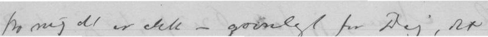tro mig det er ikke - gavnligt for Dig, det
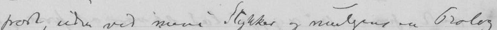træde, uden ved mine Stykker og muligvis en Prolog
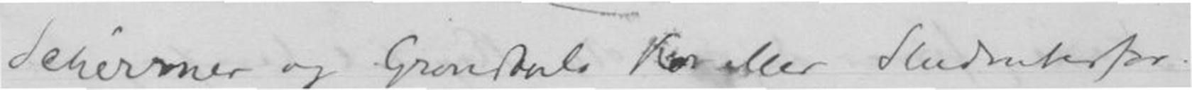Schirmer og Grøndals Kor eller Studenterfor-
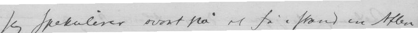Jeg spekulerer svært på at få i stand en Aften-
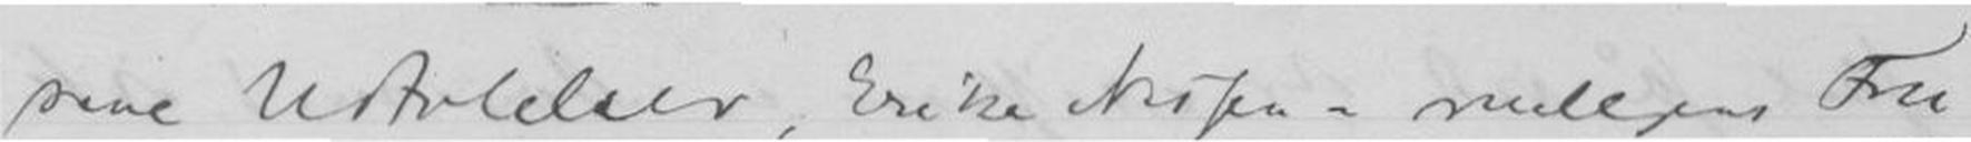sine Udtalelser, Erika Nissen - muligens Fru
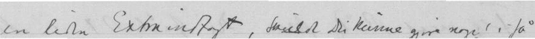en liden Extra indtægt, skulde Du kunne gjøre nap i så
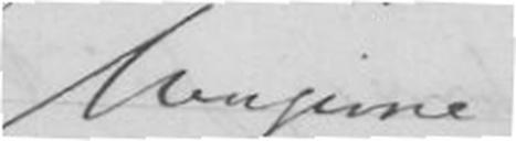hengivne
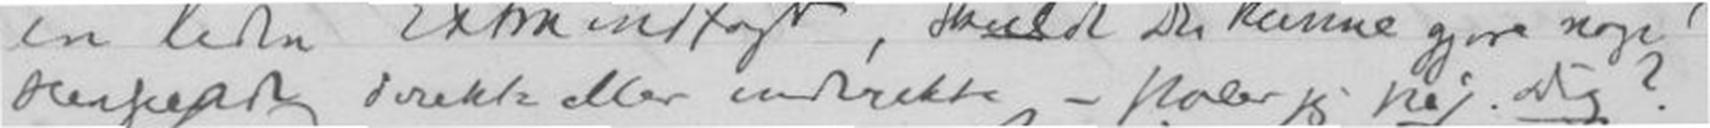Hensende direkte eller inderekte - stoler jeg på dig?
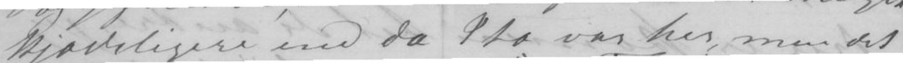kjædeligere end da I to var her, men det
